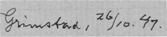Grimstad, 26/10. 47.
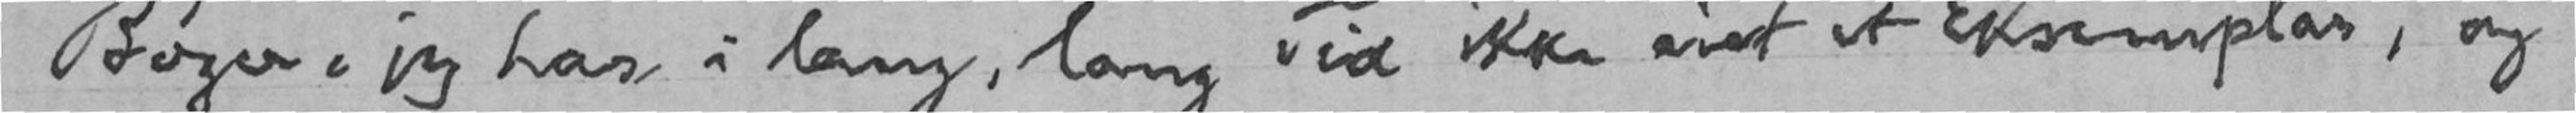Bøger, jeg har i lang, lang Tid ikke eiet et Eksemplar, og
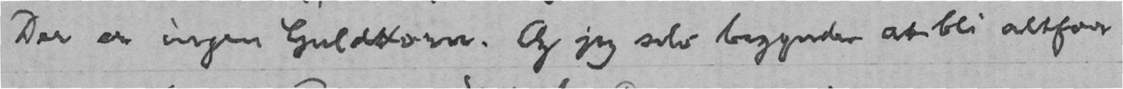Der er ingen Guldkorn. Og jeg selv begynder at bli altfor
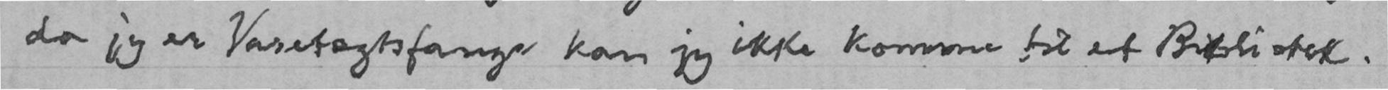da jeg er Varetægtsfange kan jeg ikke komme til et Bibliotek.
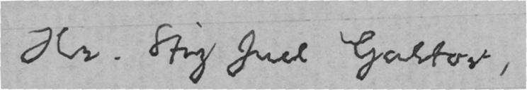Hr. Stig Juel Galtov,
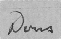Deres
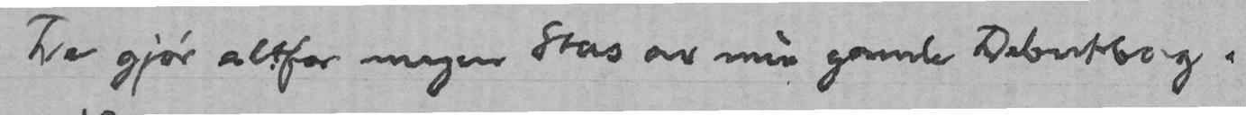De gjør altfor megen Stas av min gamle Debutbog.
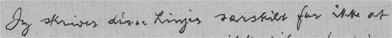Jeg skriver disse Linjer særskilt for ikke at
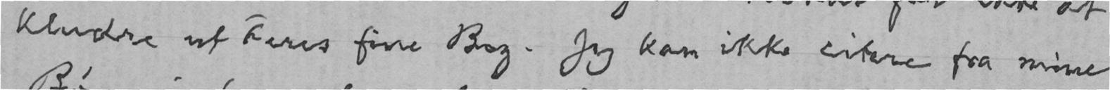kludre ut Deres fine Bog. Jeg kan ikke citere fra mine
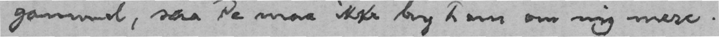gammel, saa De maa ikke bry Dem om mig mere.
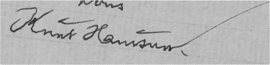Knut Hamsun.
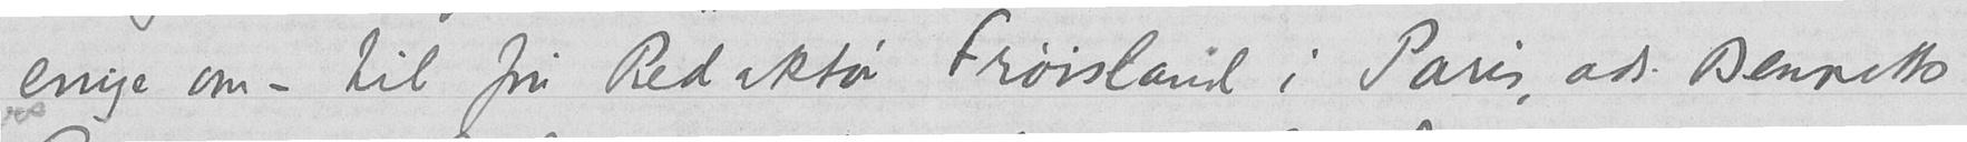enige om – til fru Redaktør Frøisland i Paris, adr. Bennets
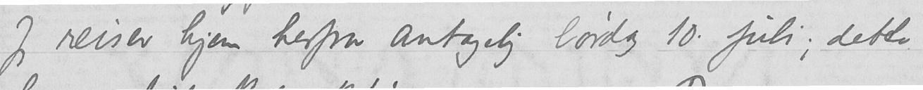Jeg reiser hjem herfra antagelig lørdag 10. juli; dette
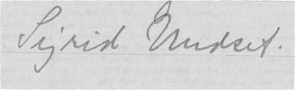Sigrid Undset.
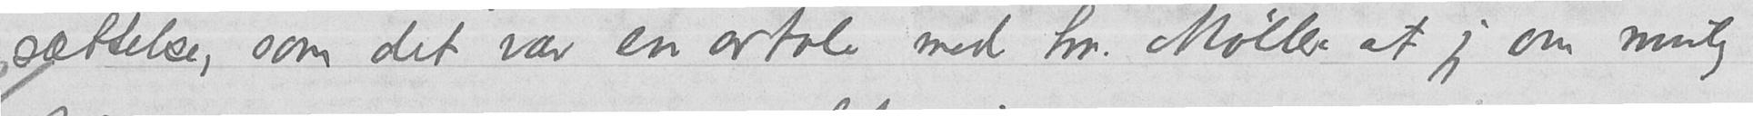sættelse, som det var en avtale med hr. Møller at jeg om mulig
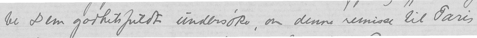be Dem godhetsfuldt undersøke, om denne remisse til Paris
